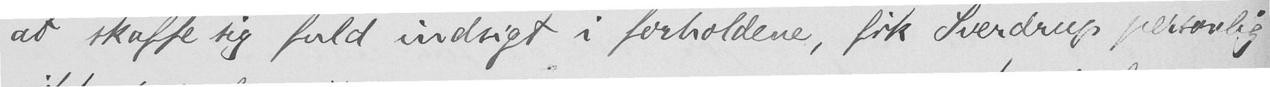at skaffe sig fuld indsigt i forholdene, fik Sverdrup personlig
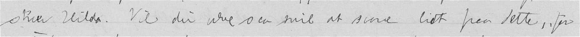skrev Hilda. Vil du være saa snil at svare lidt paa dette, for
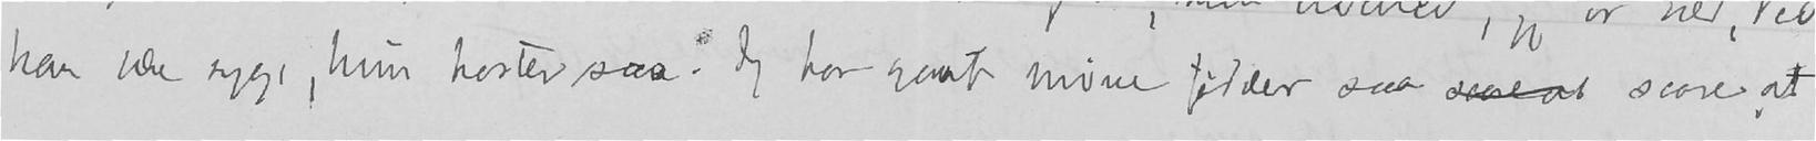kan være syg, hun hoster saa. Jeg har gaat mine fødder saa saare at saare, at
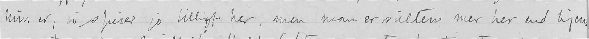hun er, vi spiser jo billigt her, men man er sulten mer her end hjem-
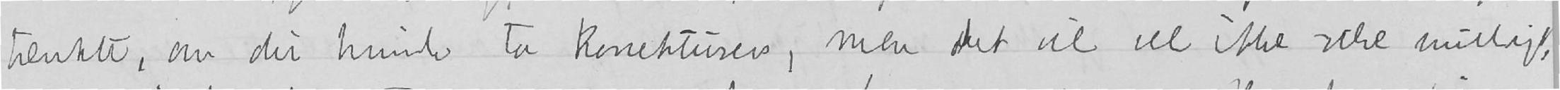tænkte, om du kunde ta korrekturen, men det vil vel ikke være muligt,
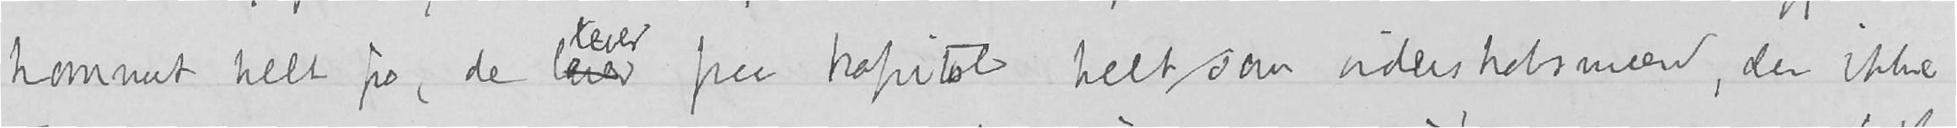kommet helt fra, de levr paa Kapitol helt som videnskabsmænd, der ikke
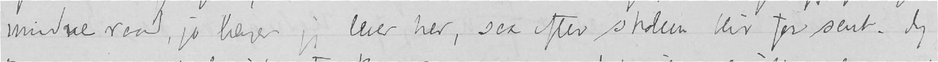mindre raad, jo længer jeg lever her, saa efter skolen blir for sent. Jeg
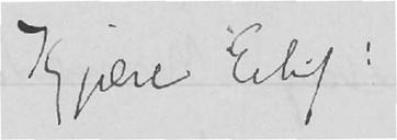Kjære Eilif!
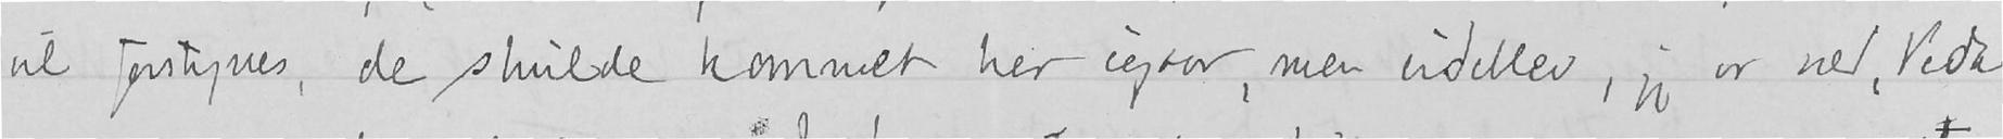vil forstyrres, de skulde kommet her igaar, men udeblev, jeg er ræd, Veda
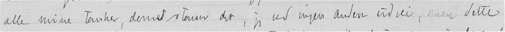alle mine tanker, dermed stanser det, jeg ved ingen anden udvei, men dette
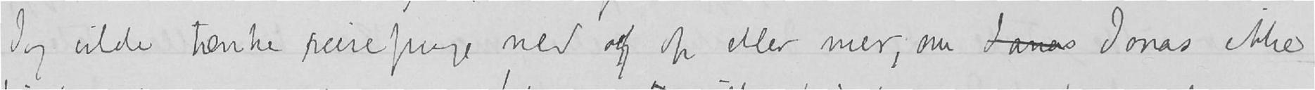Jeg vilde tænke reisepenge ned og op eller mer, om Janas Jonas ikke
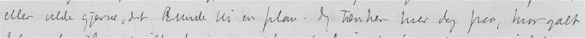eller vilde gjerne, det kunde bli en plan. Jeg tænker hver dag paa, hvor galt
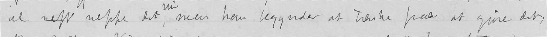vel nept neppe det, men han begynder at tænke paa at gjøre det;
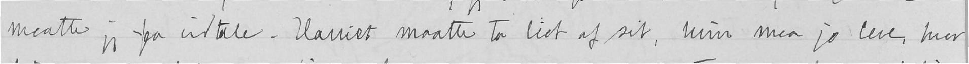maatte jeg faa udtale. Harriet maatte ta lidt af sit, hun maa jo leve, hvor
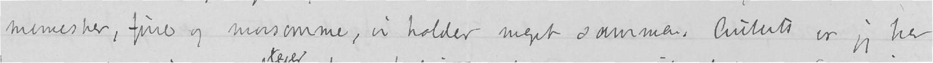mennesker, fine og morsomme, vi holder meget sammen. Auberts er jeg her
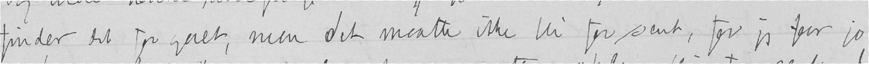finder det for galt, men det maatte ikke bli for sent, for jeg faar jo
